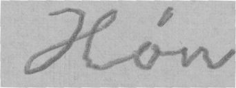Høn
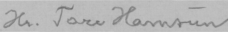Hr. Tore Hamsun
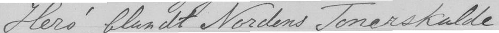Hero' blandt Nordens Tonerskalde
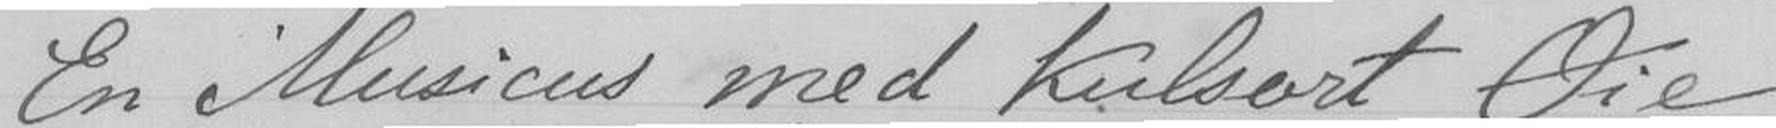En Musicers med Kulsort Øie
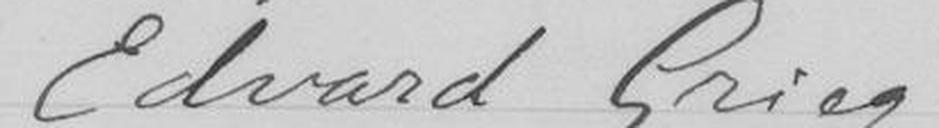Edvard Grieg
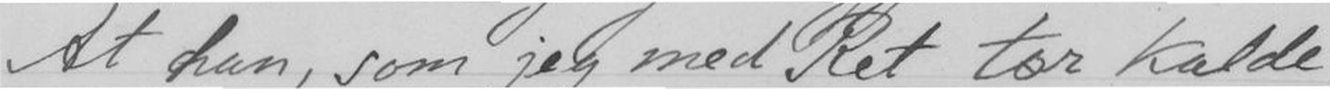At han, som jeg med Ret tør kalde
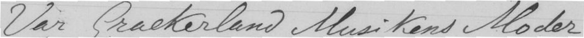Vor Graekerland Musikens Moder
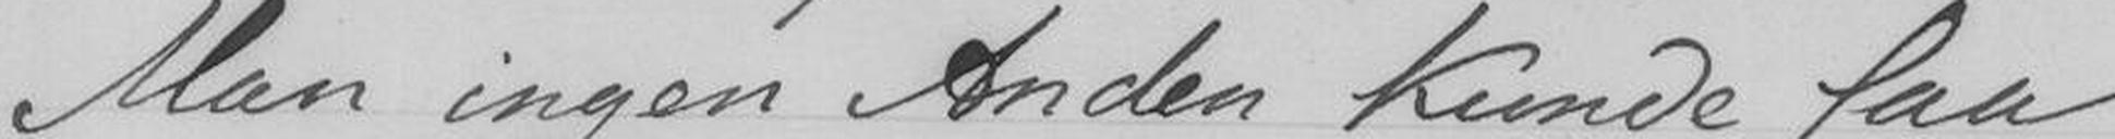Man ingen Anden Kunde faa
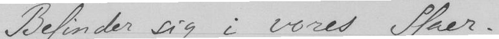Befinder sig i vores Haer.
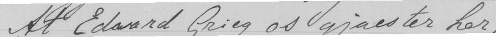At Edvard Grieg os gjaester her,
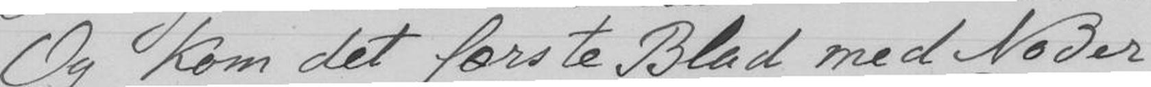Og kom det første Blad med Noder
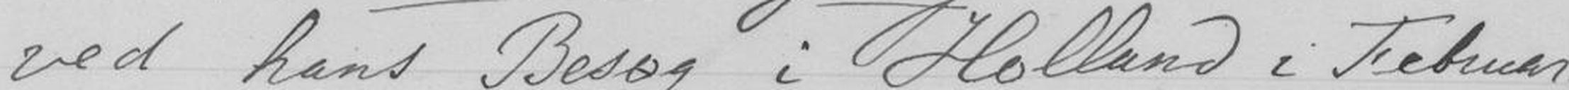ved hans Besøg i Holland i Februar
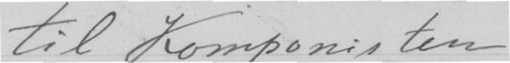til Komponisten
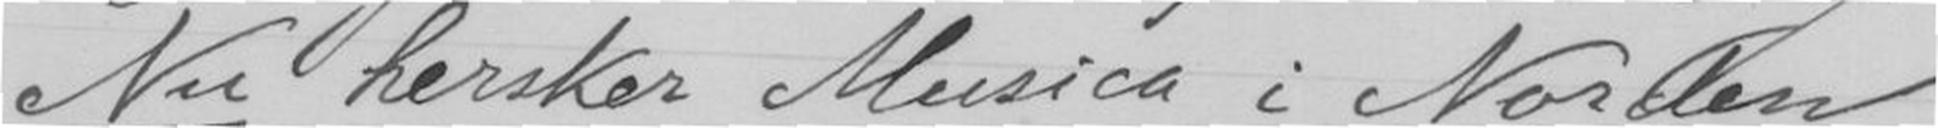Nu hersker Musica i Norden
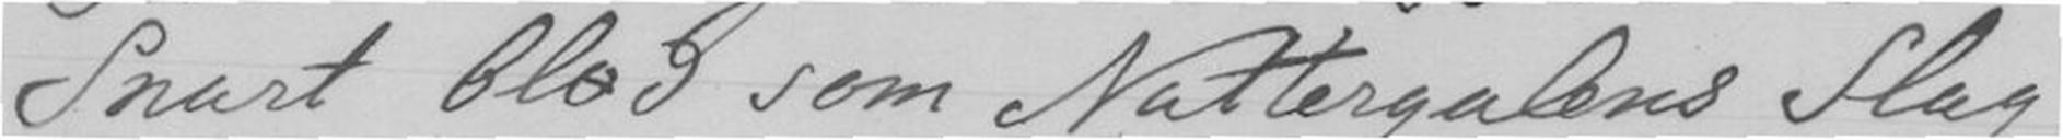Snart blød som Natergalens Slag.
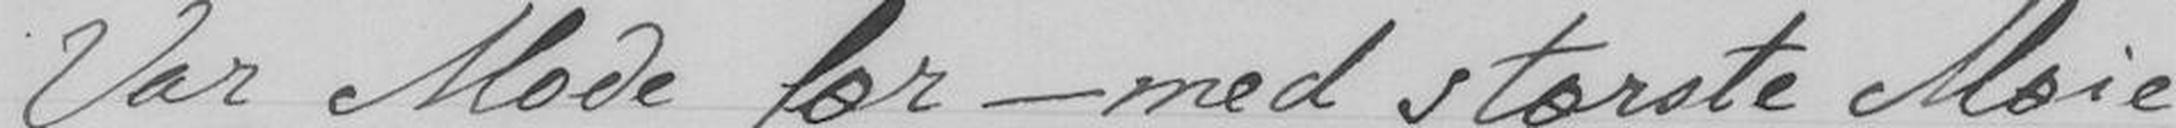Var Mode før - med største Møie
